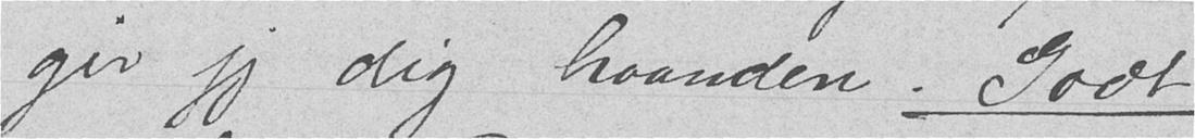gir jeg dig haanden. Godt
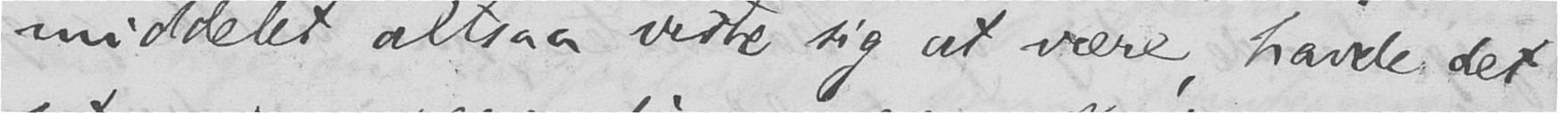middelet altsaa viste sig at være, havde det
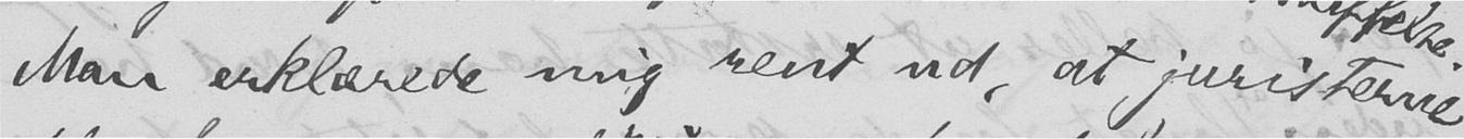Man erklærede mig rent ud, at juristerne
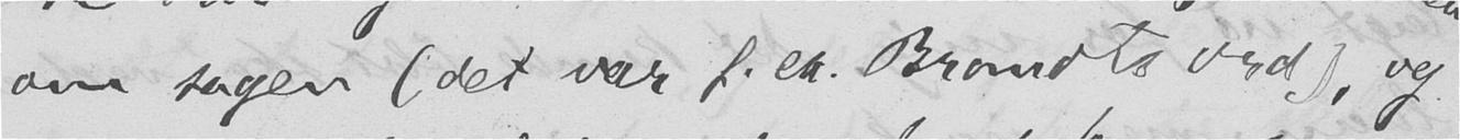om sagen (det var f.ex. Brandts ord), og
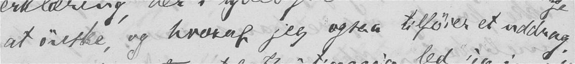at ønske, og hvoraf jeg ogsaa tilføier et uddrag.
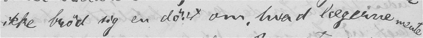ikke brød sig en døit om, hvad lægerne mente
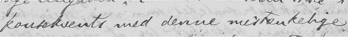konsekvents med denne mistænkelige
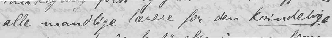alle mandlige lærere for den kvindelige
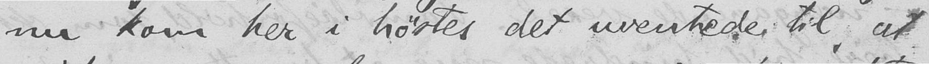nu kom her i høstes det uventæde til, at
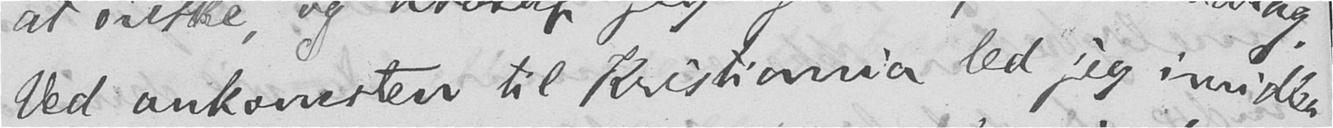Ved ankomsten til Kristiania, led jeg imidler-
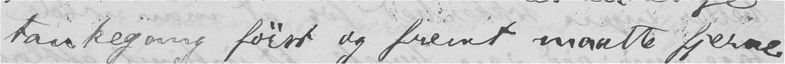tankegang først og fremt maatte fjerne
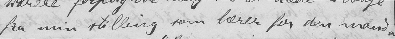fra min stilling som lærer for den mand-
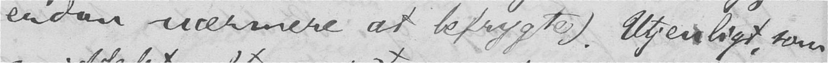endnu nærmere at befrygte). Utjenligt, som
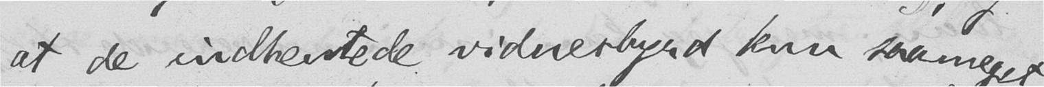at de indhentede vidnesbyrd kun saameget
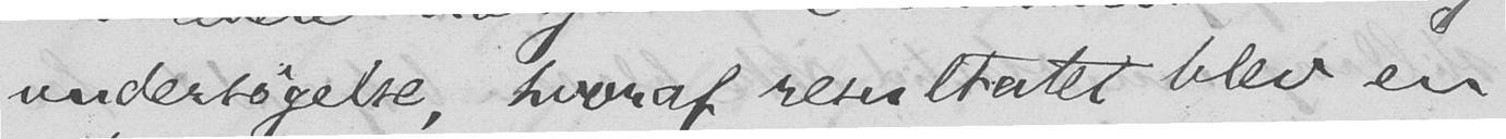undersøgelse, hvoraf resultatet blev en
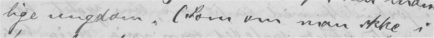lige ungdom. (Som om man ikke i
